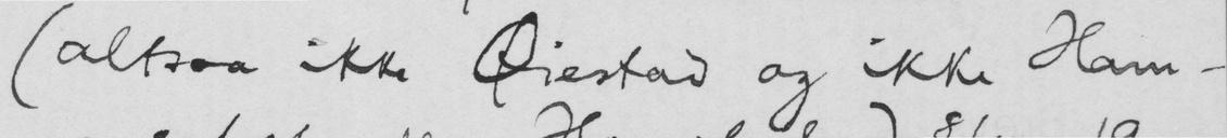(altsaa ikke Øiestad og ikke Ham-
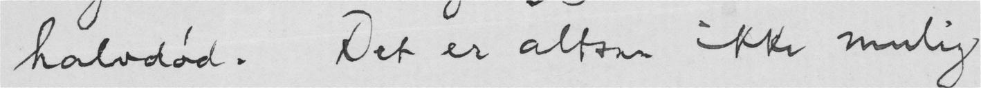halvdød. Det er altsaa ikke mulig
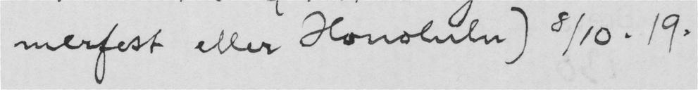merfest eller Honolulu) 8/10. 19.
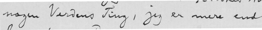nogen Verdens Ting, jeg er mere end
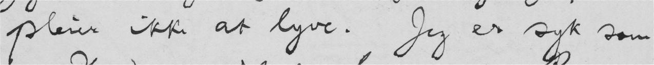pleier ikke at lyve. Jeg er syk som
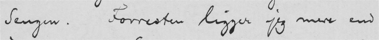Sengen. Forresten ligger jeg mere end
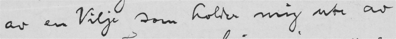av en Vilje som holder mig ute av
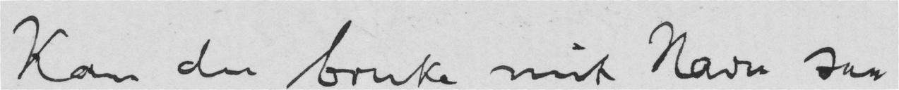Kan du bruke mit Navn saa
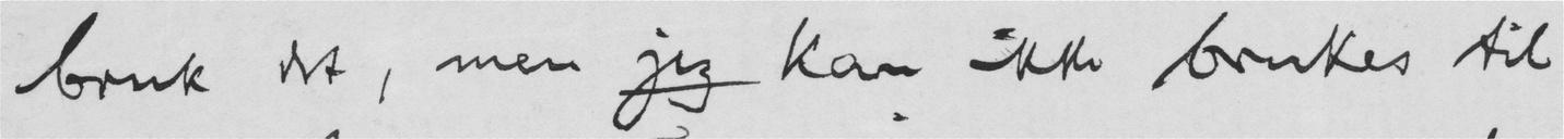bruk det, men jeg kan ikke brukes til
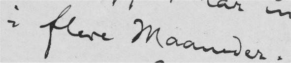i flere Maaneder.
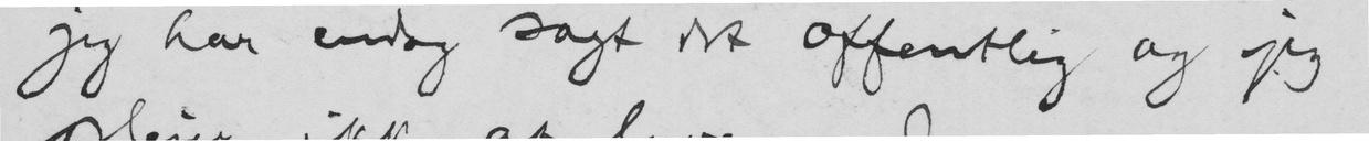jeg har endog sagt det offentlig og jeg
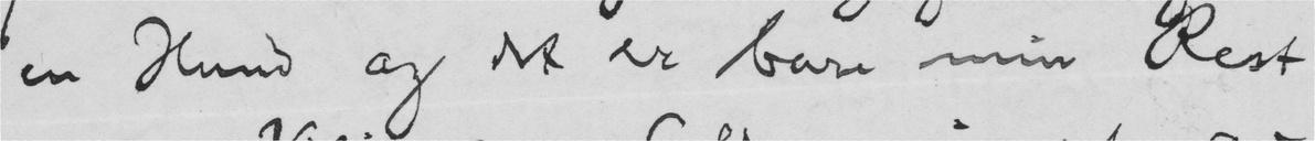en Hund og det er bare min Rest
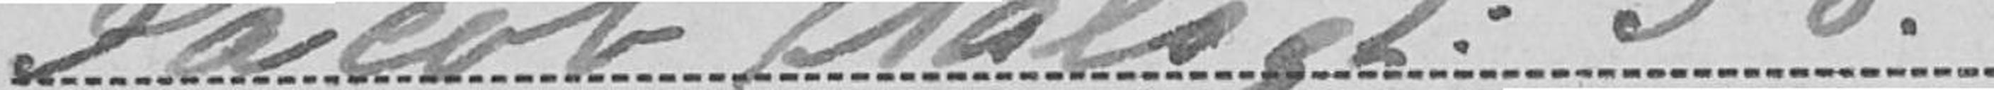Jacob Aalsgt: 25.
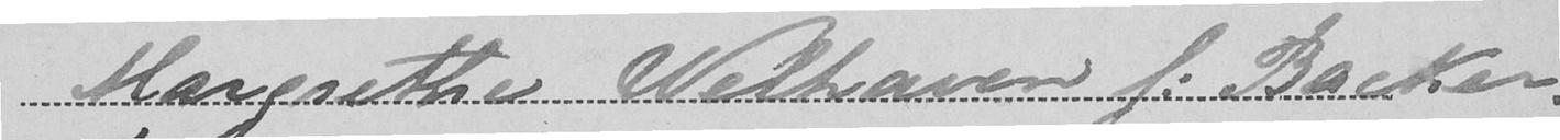Margrethe Welhaven f: Backer.
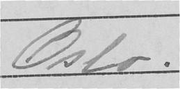Oslo.
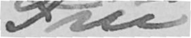Fru
